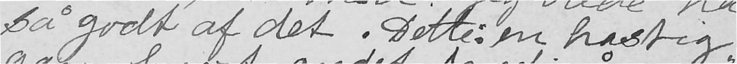så godt af det. Dette: en hastig
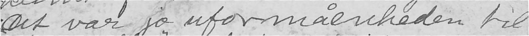Det var jo uformåenheden til
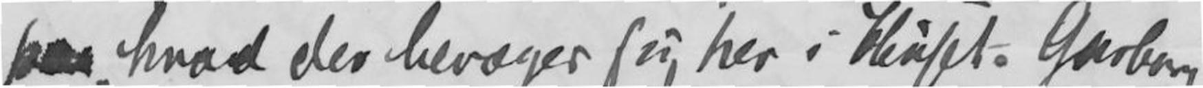paa, hvad der bevæger sig her i Huset. Garborg
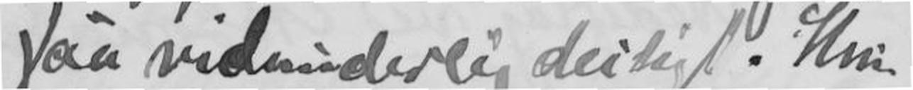saa vidunderlig deiligt. Hun
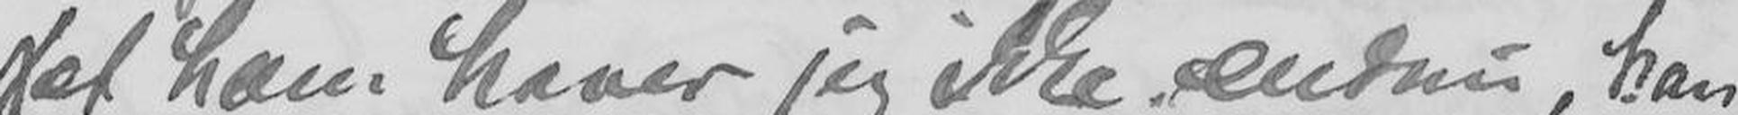set ham haver jeg ikke ændnu, han
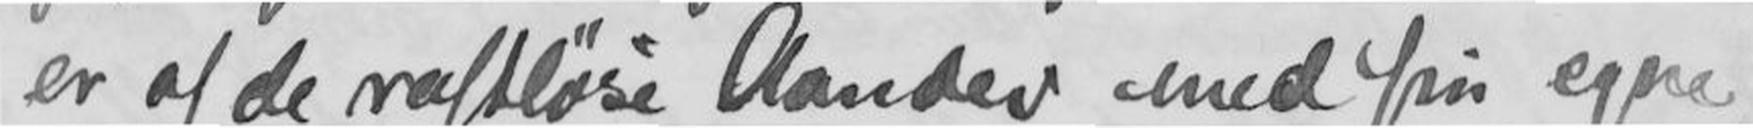er af de rastløse Aander med sin egen
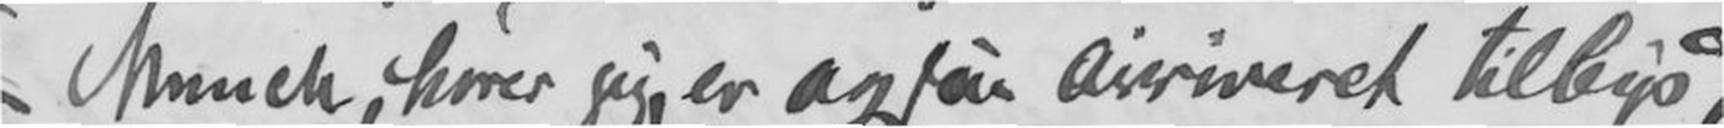Munch, hører jeg, er ogsaa arriveret tilbys,
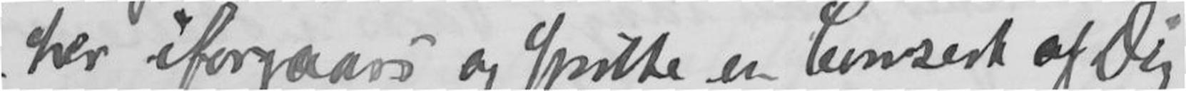her iforgaars og spilte en Consert af Dig
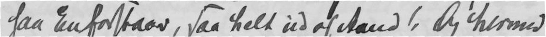saa En forstaar, saa helt ud af Aand! Og hermed
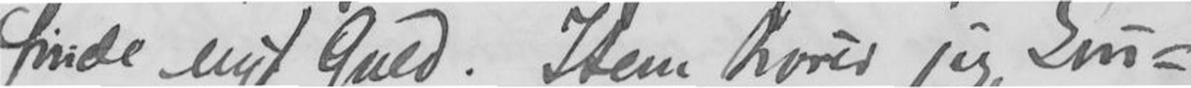finde nyt Guld. Item hører jeg, Sin-
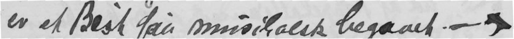er et Best saa musikalsk begavet - og
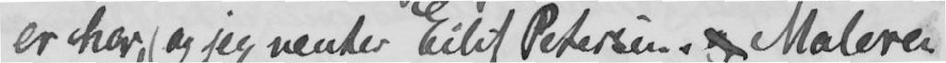er her, og jeg venter Eilif Petersen og Maleren
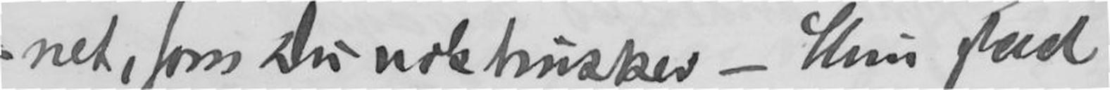net, som Du nok husker - Hun sad
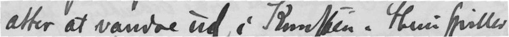atter at vandre ud i Kunsten. Hun spiller
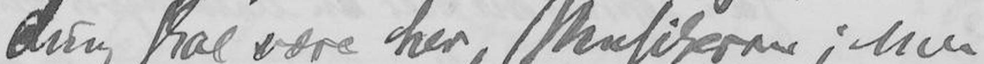ding skal være her, Musikeren; men
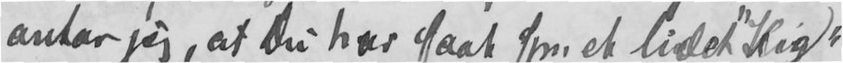antar jeg, at Du har faat som et lidet "Kig"
